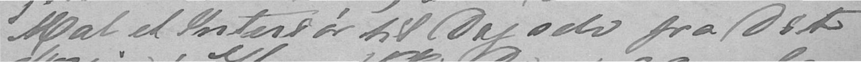Mal et Interiør til Dig selv fra Dit
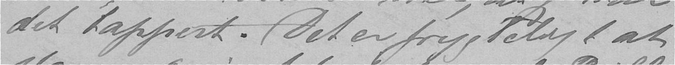det tappert. Det er frygteligt at
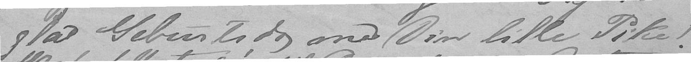glad Geburtsdag med Din lille Pike!
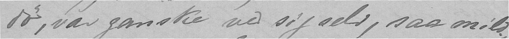dø, var ganske ved sig selv, saa mild
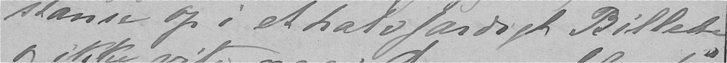stanse op i et halvfærdigt Billede
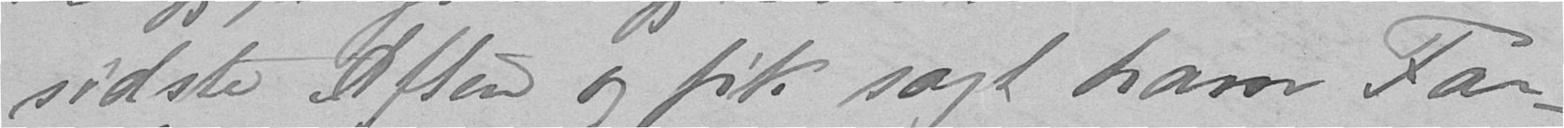sidste Aften og fik sagt ham Far-
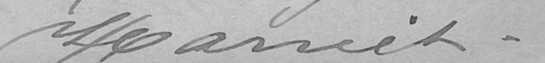Harriet.
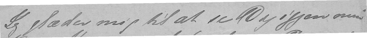Jeg glæder mig til at se Dig igjen min
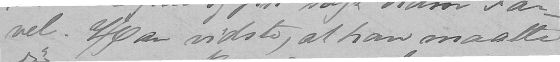vel. Han vidste, at han maatte
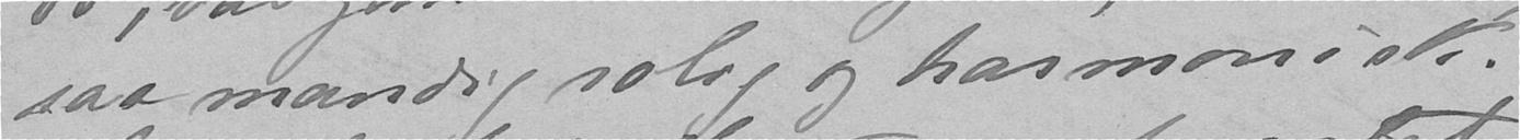saa mandig rolig og harmonisk.
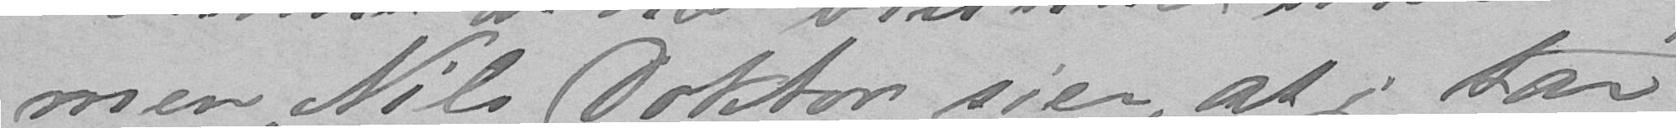men, Nils Doktor sier, at jeg tar
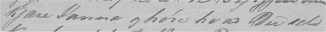kjære Janna og høre hvad Du selv
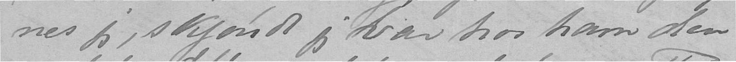nes jeg, skjøndt jeg var hos ham den
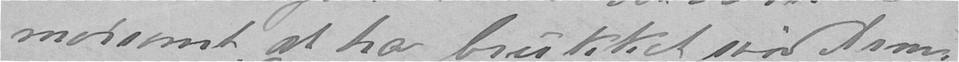morsomt at ha brukket sin Arm,
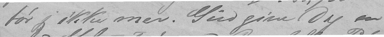tør jeg ikke mer. Gud give Dig en
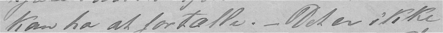kan ha at fortælle. - Det er ikke
